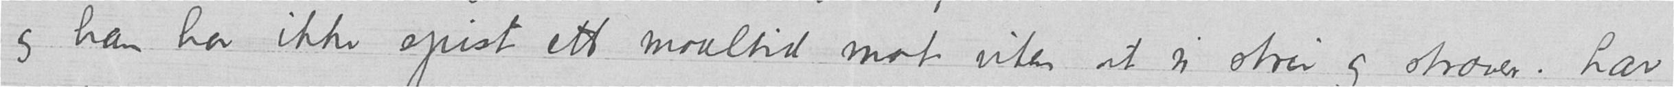og han har ikke spist ett maaltid mat uten at vi strir og stræver. Lar
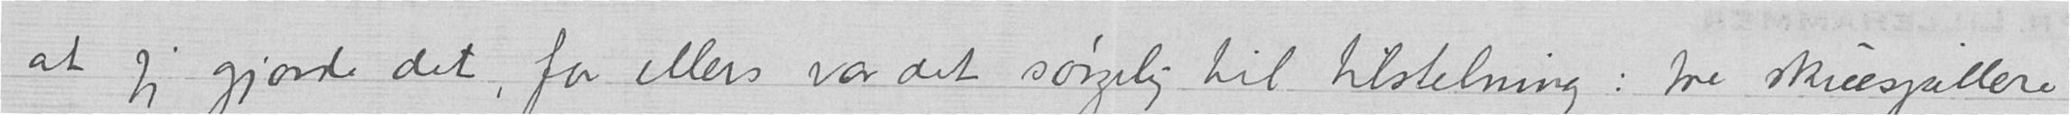at jeg gjorde det, for ellers var det sørgelig til tilstelning: tre skuespillere
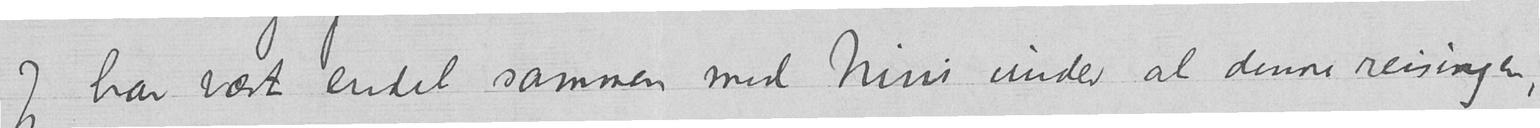Jeg har vært endel sammen med Nini under al denne reisingen,
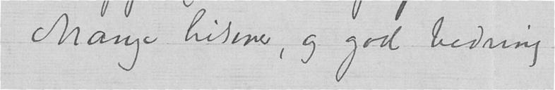Mange hilsener, og god bedring
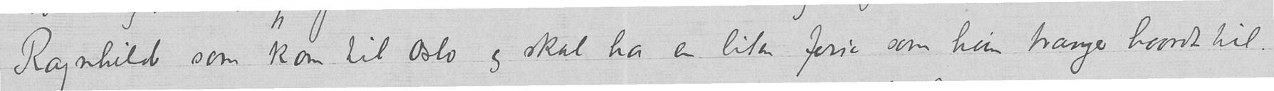Ragnhild som kom til Oslo og skal ha en liten ferie som hun trænger haardt til.
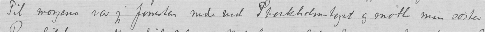Til morgens var jeg forresten nede ved Stockholmstoget og møtte min søster
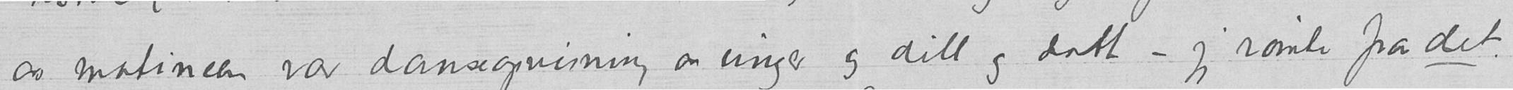av matineen var danseopvisning av unger og ditt og datt – jeg rømle fra det .
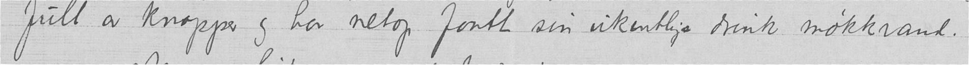full av knopper og har netop faatt sin ukentlige drink møkkvand.
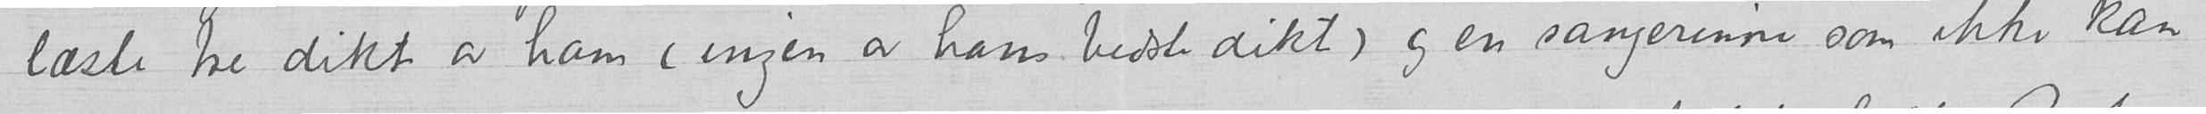læste tre dikt av ham (ingen av hans bedste dikt) og en sangerenne som ikke kan
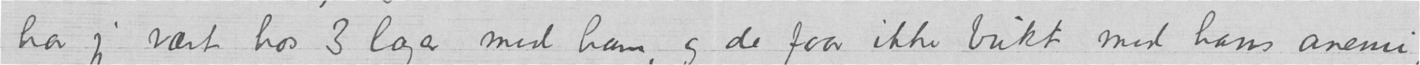har jeg vært hos 3 læger med ham, og de faar ikke bukt med hans anemi,
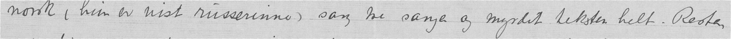norsk (hun er vist russerinne) sang tre sanger og myrdet teksten helt. Resten
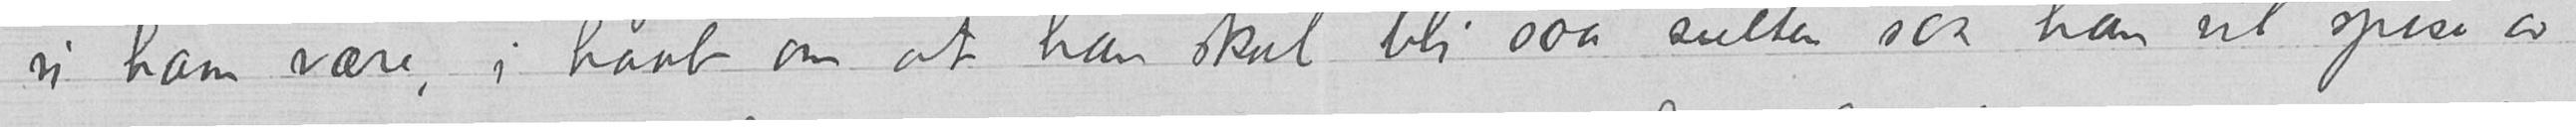vi ham være, i haab om at han skal bli saa sulten saa han vil spise av
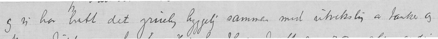og vi har hatt det gruelig hyggelig sammen med utveksling av tanker og
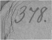378.
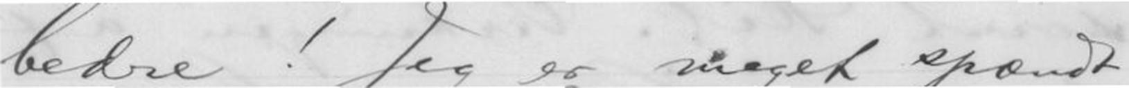bedre! Jeg er meget spændt
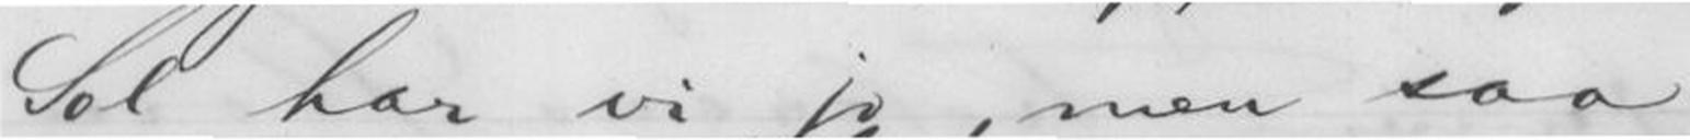Sol har vi jo, men saa
